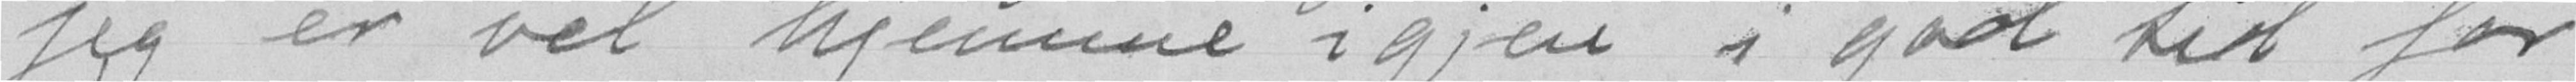jeg er vel hjemme igjen i god tid før
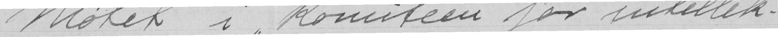Møtet i Komiteen for intellek-
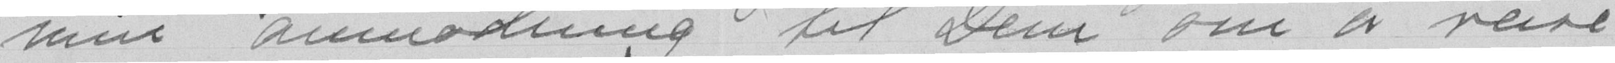min anmodning til Dem om å reise
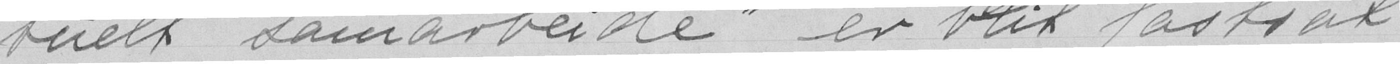tuelt samarbeide er fastsat
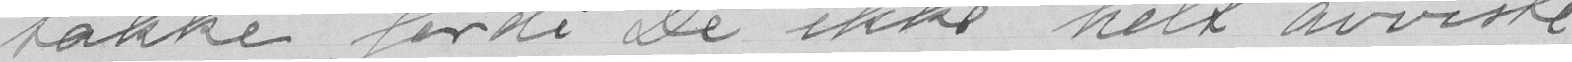takke fordi De ikke helt avviste
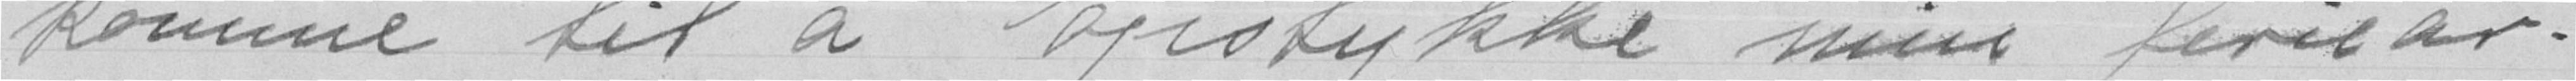komme til å opstykke min feriear-
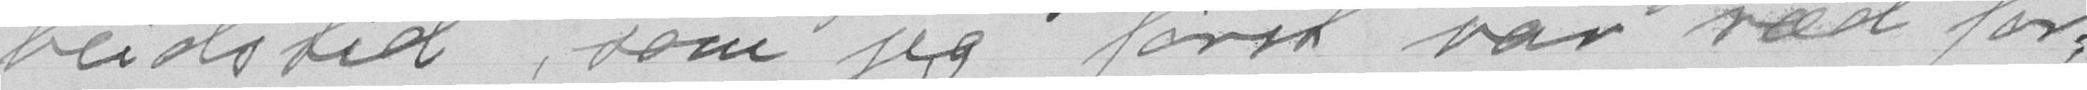beidstid, som jeg først var ræd for,
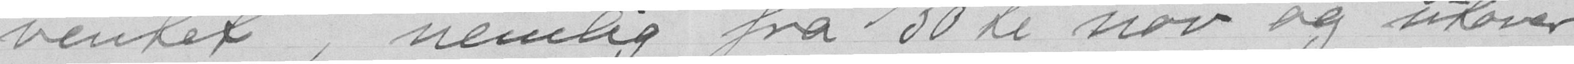ventet, nemlig fra 30te nov og utover
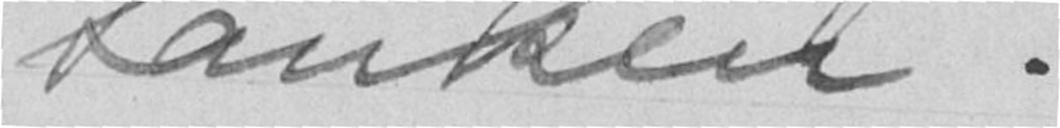tanken.
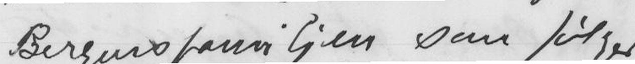Bergensfamiljen som følger
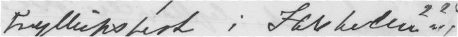bryllupsfest i Stokholm???
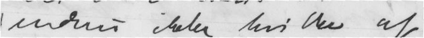endnu ikke hvilke af
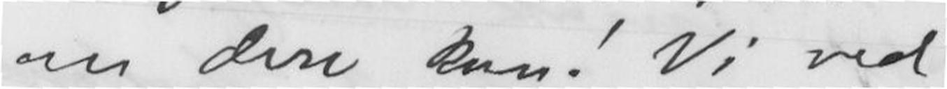om dere kom! Vi ved
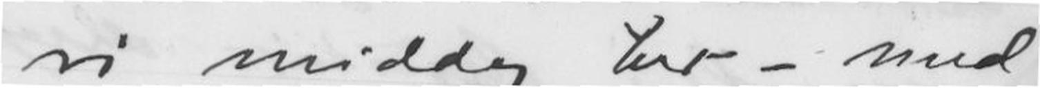vi middag her - med
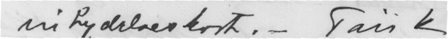inbydelseskort. - Tänk
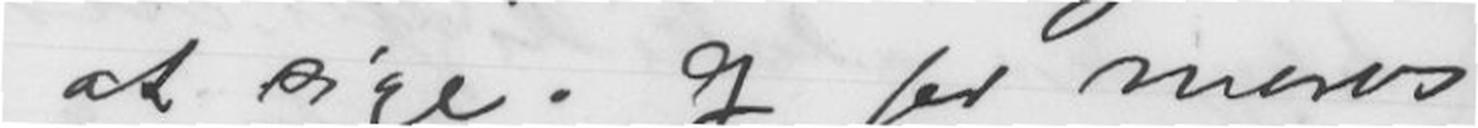at sige. Og for moros
